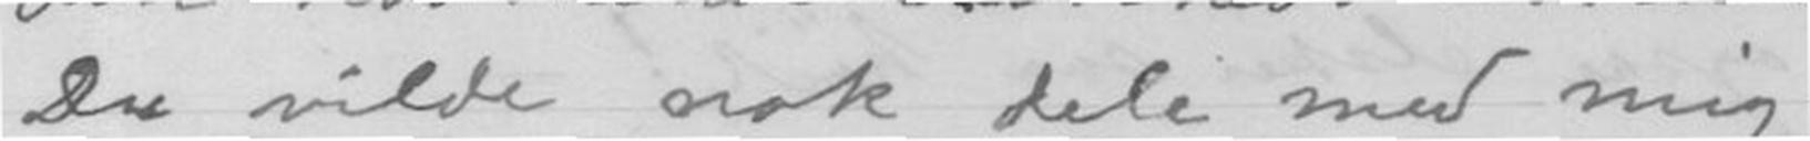Du vilde nok dele med mig
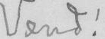Vend!
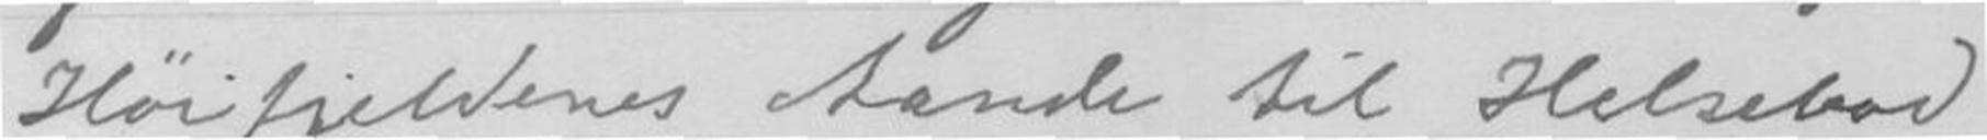Høifjeldenes Aande til Helsebod
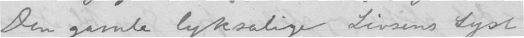Den gamle lyksalige Livsens Lyst
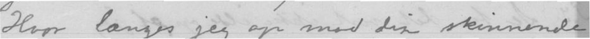Hvor længes jeg op mod din skinnende
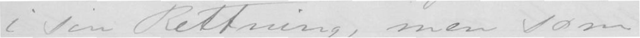i sin Rettning, men som
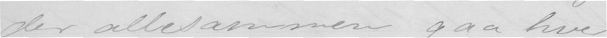der allesammen gaa hver
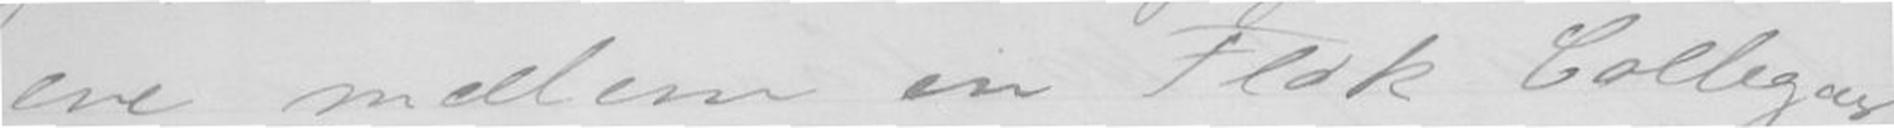ene mellem en Flok Colleger
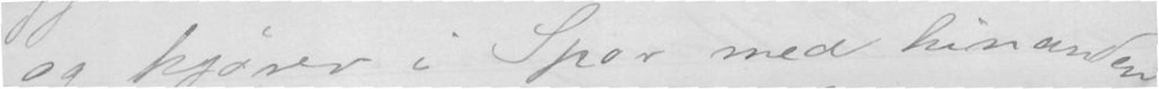og kjører i Spor med hinanden
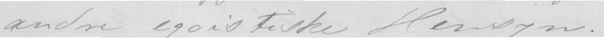andre egoistiske Hensyn. -
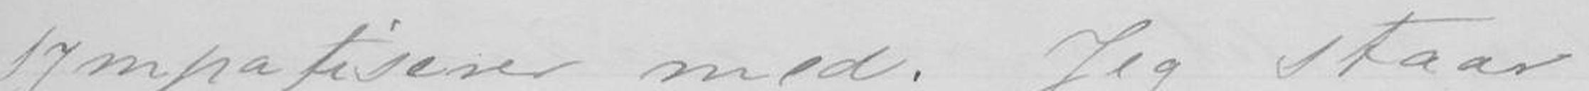sympatiserer med. Jeg staar
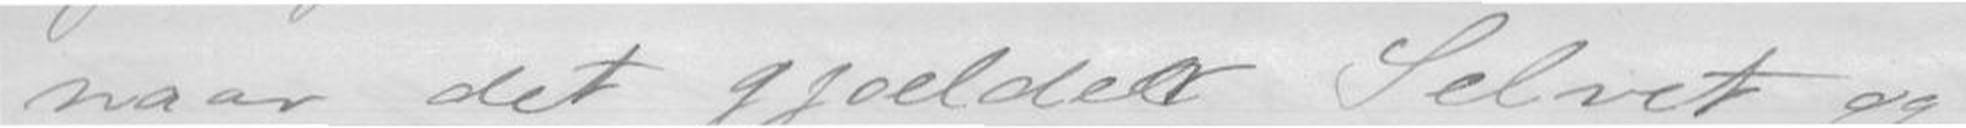naar det gjælder Selvet og
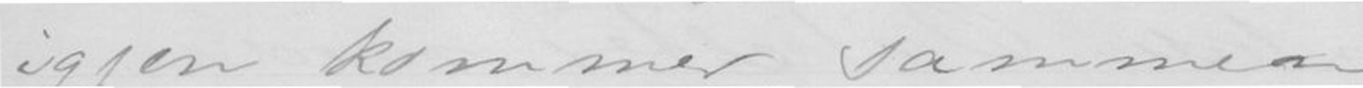igjen kommer sammen
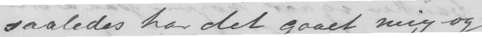saaledes har det gaaet mig og
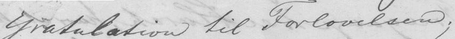Gratulation til Forlovelsen;
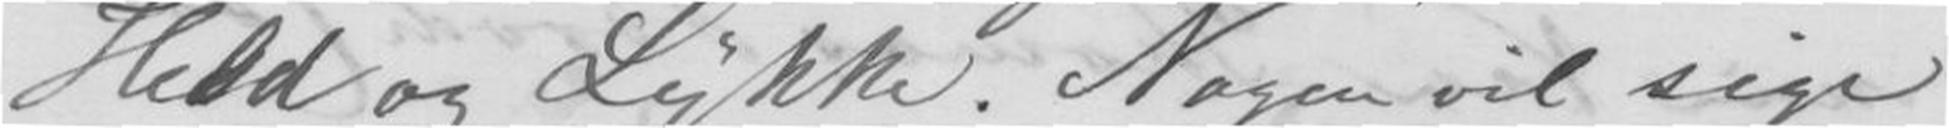Held og Lykke. Nogen vil sige
